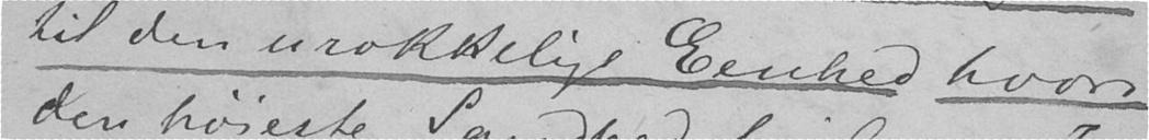til den urokkelige Eenhed hvori
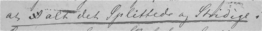at alt det Splittede og Stadige i
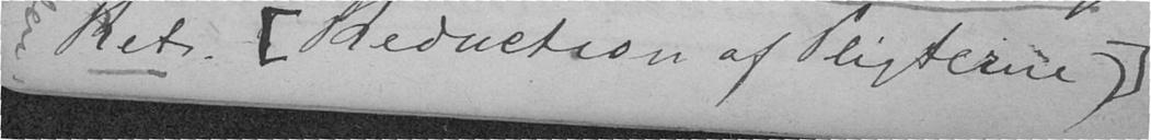Ret. [Deduction af Pligterne)]
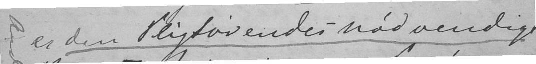er den Pligtøvendes nødvendige
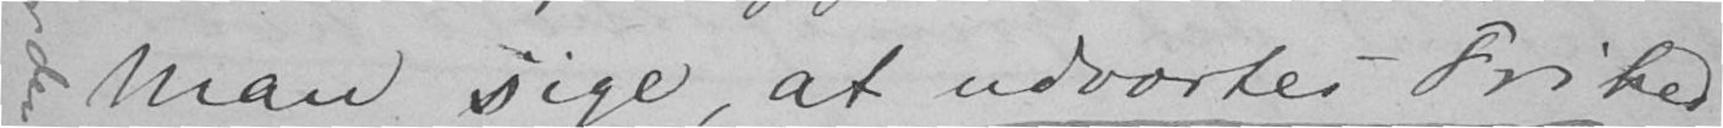man sige, at udvortes Frihed
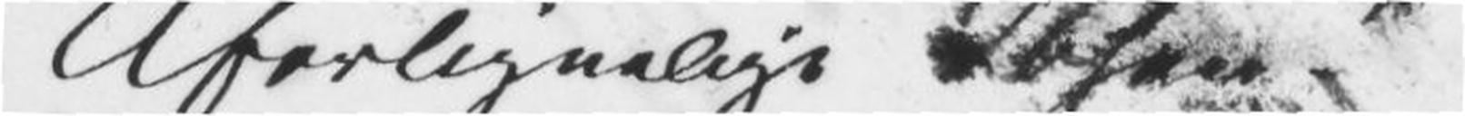Uforlignelige Ibsen!
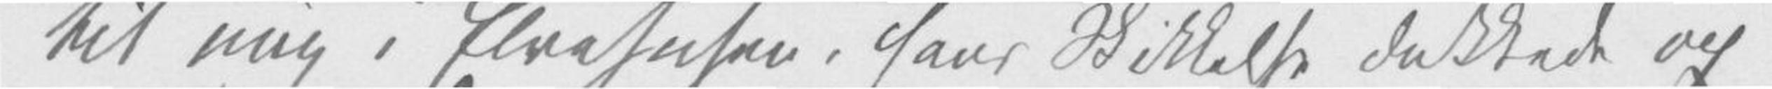til mig i Elvesusen, hans Skikkelse dukkede op
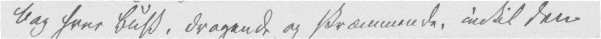bag hver Busk, dragende og skræmmende, indtil den
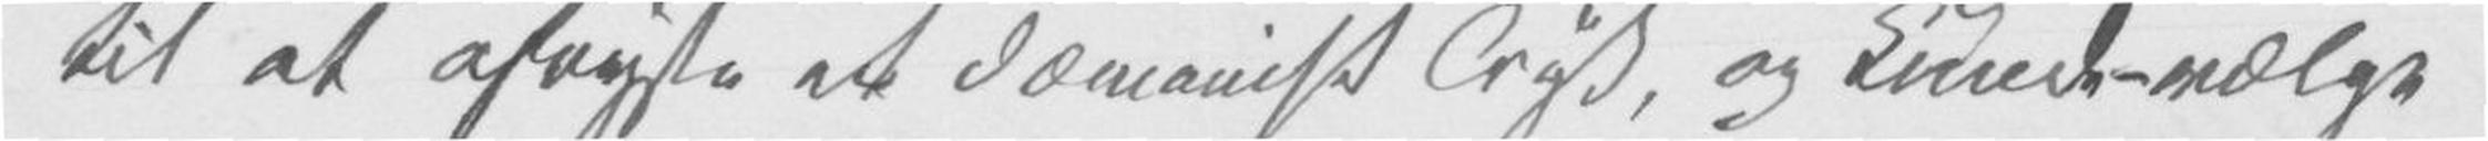til at afryste et dæmonisk Tryk, og kunde – «vælge
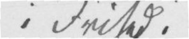i Frihed».
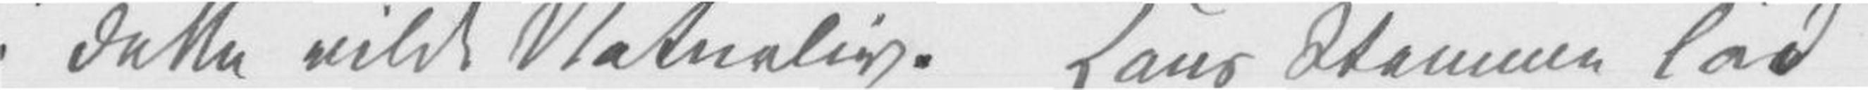i dette vilde Naturliv. Hans Stemme lød
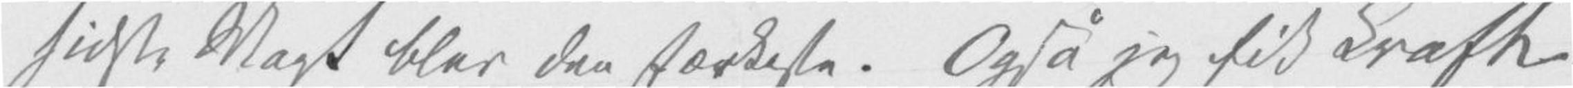sidste Magt blev den stærkeste. Også jeg fik Kraft
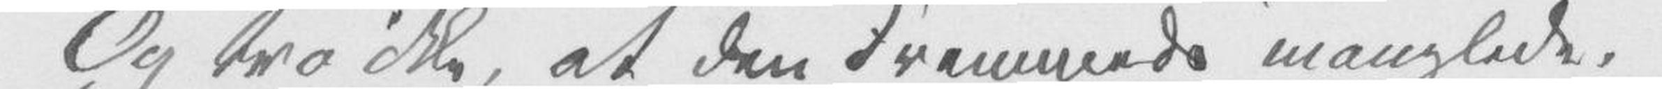Og tro ikke, at «den Fremmede» manglede
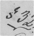§ 32.
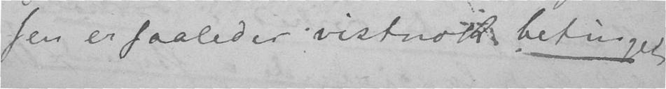en er saaledes vistnok betinget
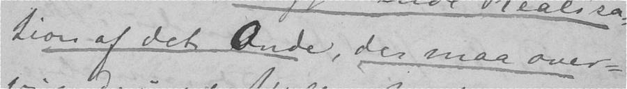tion af det Onde, der maa over-
